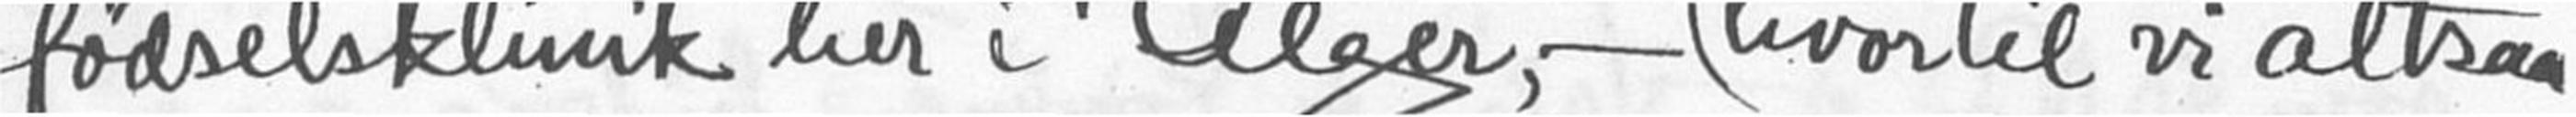fødselsklinik her i Alger, - (hvortil vi altsaa
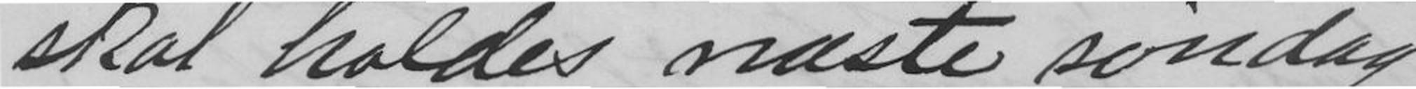skal holdes næste søndag
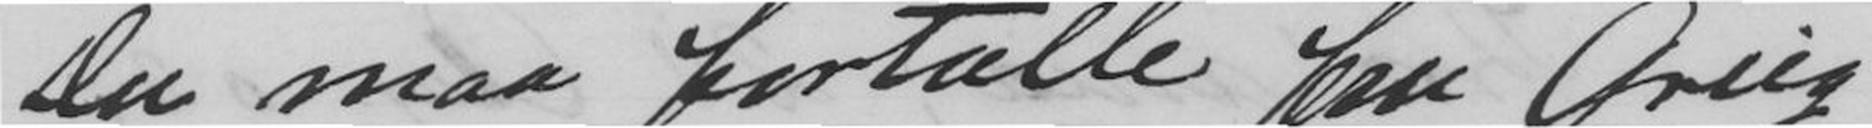Du maa fortælle fru Grieg,
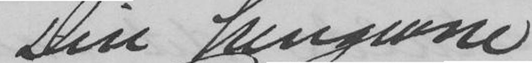Din hengivne
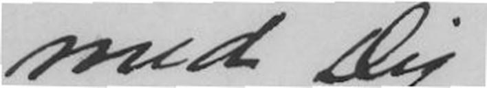med Dig
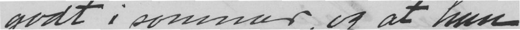godt i sommer, og at hun
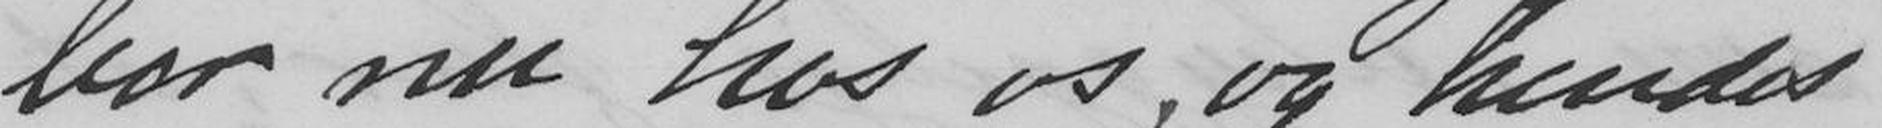bor nu hos os, og hendes
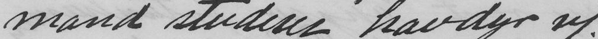mand studerer havdyr v/.
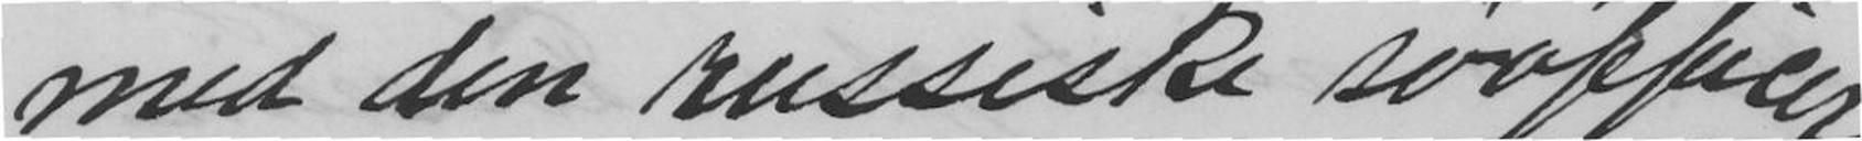med den russiske søofficer.
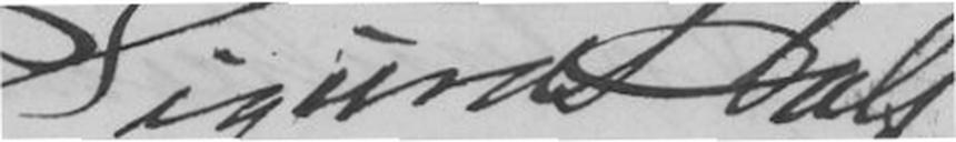Sigurd Hals
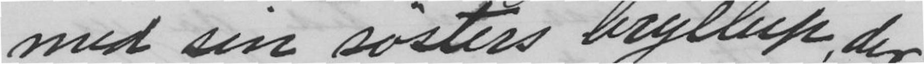med sin søsters bryllup, der
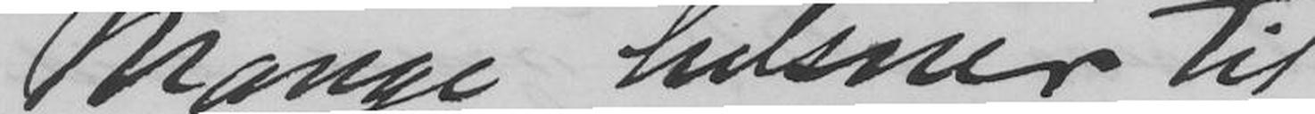Mange hilsner til
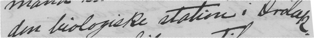den biologiske station i Drøbak.
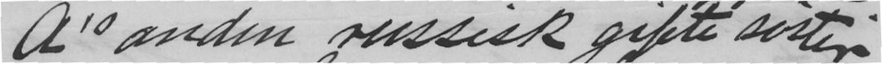A's anden russisk gifte søster
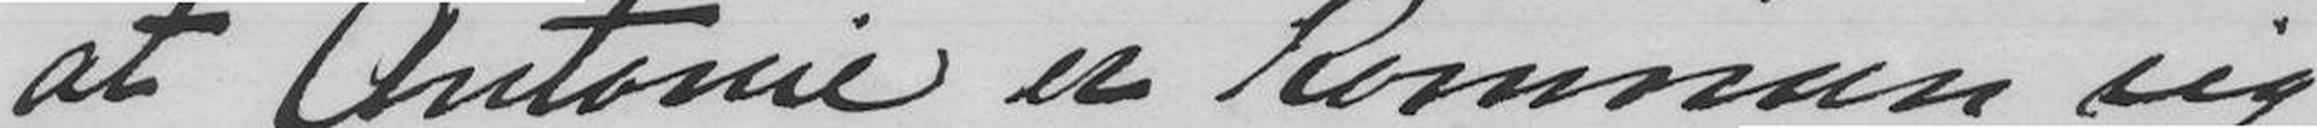at Antonie er kommen sig
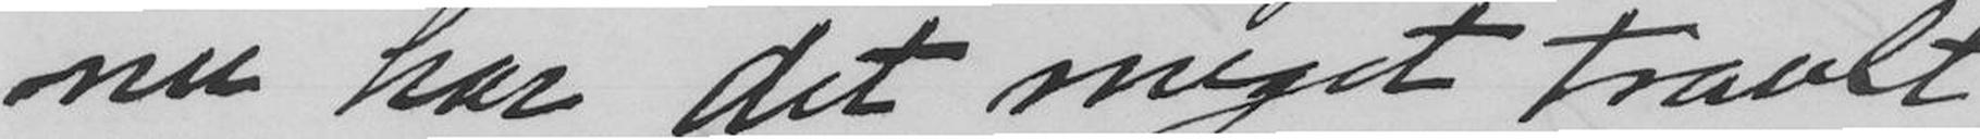nu har det meget travlt
